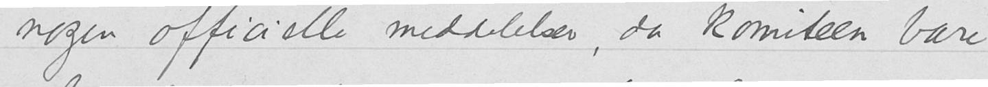nogen officielle meddelelser, da komiteen bare
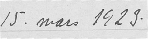15. mars 1923.
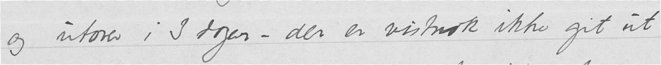og utover i 3 dager - der er vistnok ikke git ut
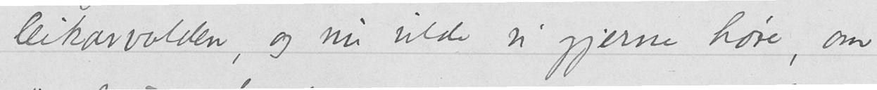leikarvolden, og nu vilde vi gjerne høre, om
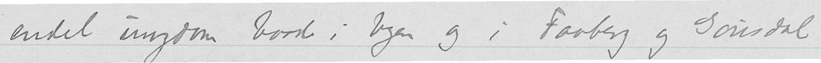endel ungdom baade i byen og i Faaberg og Gousdal
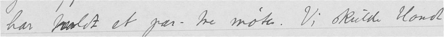har holdt et par – tre møter. Vi skulde blandt
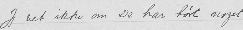Jeg vet ikke om De har hørt noget
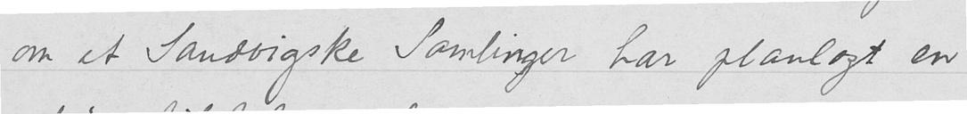om at Sandvigske Samlinger har planlagt en
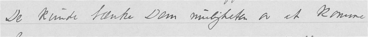De kunde tænke Dem muligheten av at komme
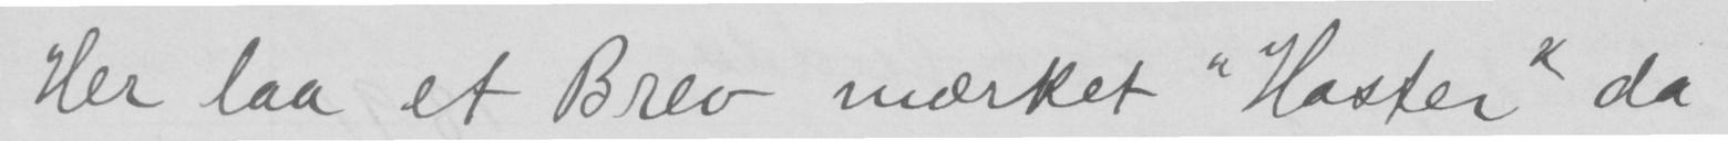Her laa et Brev mærket «Haster» da
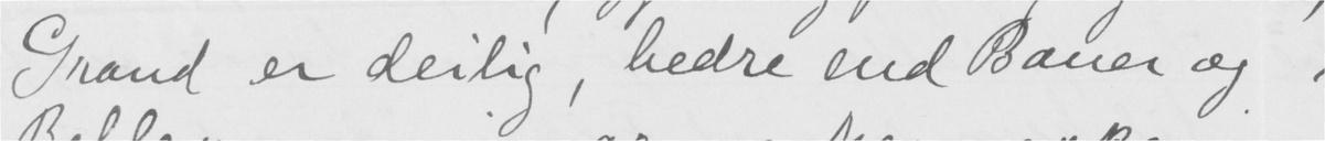Grand er deilig, bedre end Baner og
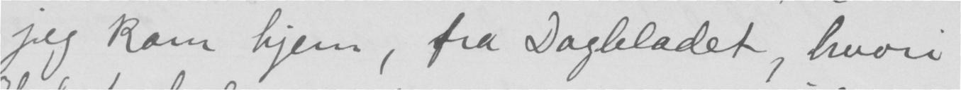jeg kom hjem, fra Dagbladet, hvori
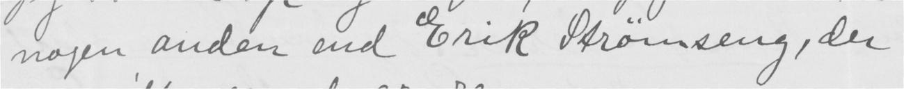nogen anden end Erik Strømseng, der
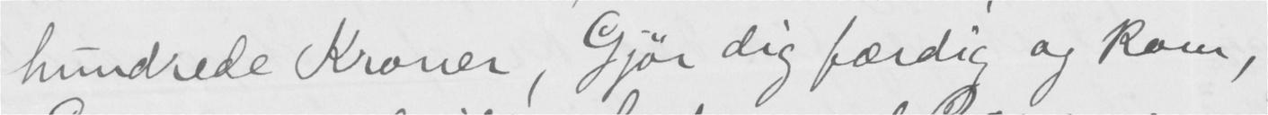hundrede Kroner, Gjør dig færdig og kom,
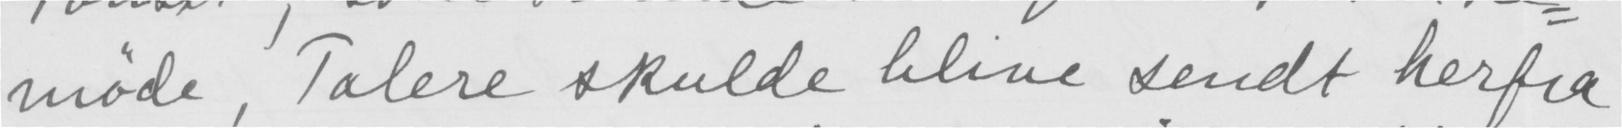møde, Talere skulde blive sendt herfra.
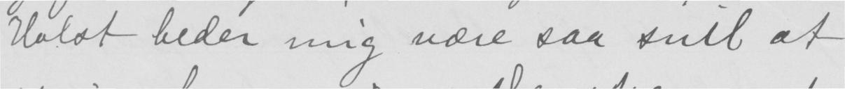Holst beder mig være saa snil at
